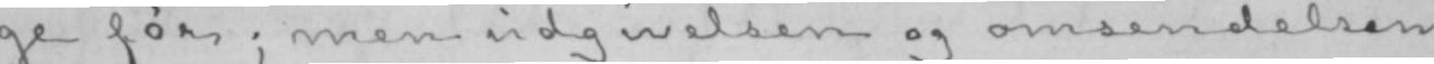ge før; men udgivelsen og omsendelsen
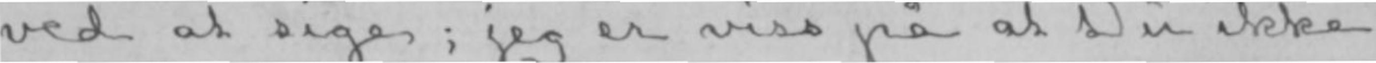ved at sige; jeg er viss på at Du ikke
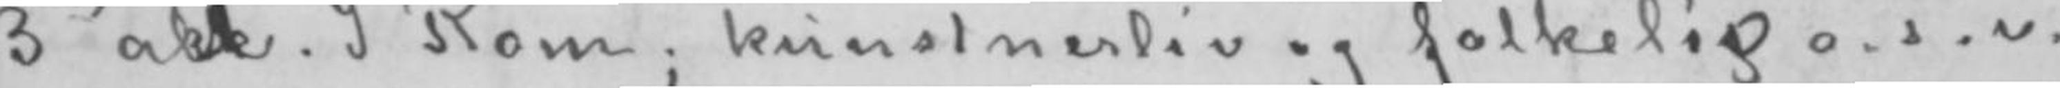3die akt. I Rom, kunstnerliv og folkeliv o. s. v.
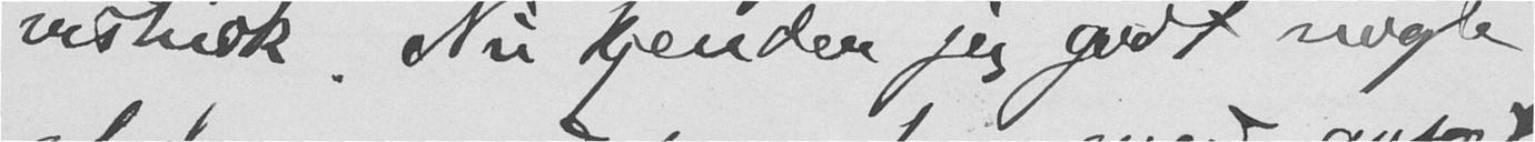vistnok. Nu kjender jeg godt nogle
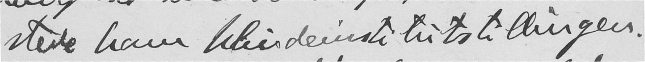stede ham blindeinstitutstillingen.
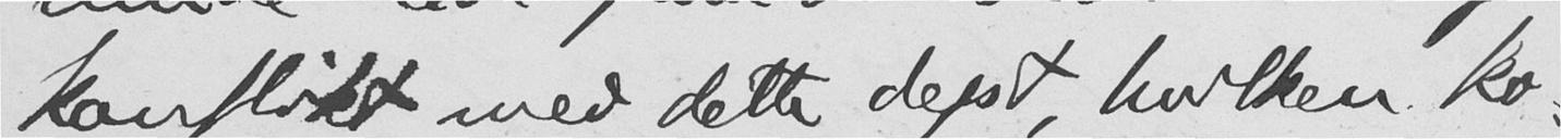konflikt med dette dept, hvilken ko-
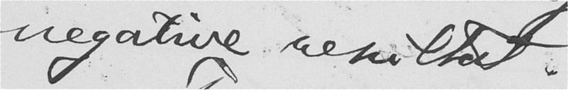negative resultat.
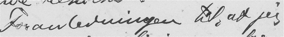Foranledningen til, at jeg
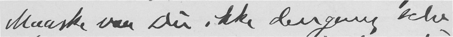Maaske var Du ikke dengang selv
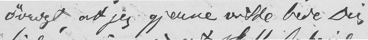øvrigt, at jeg gjerne vilde bede Dig
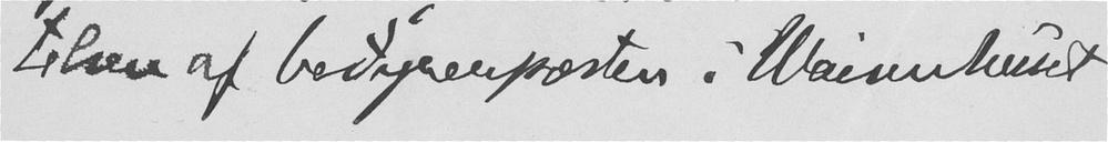telsen af bestyrerposten i Waisenhuset
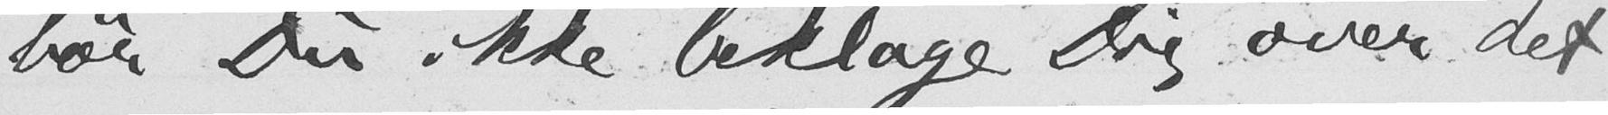bør Du ikke beklage Dig over det
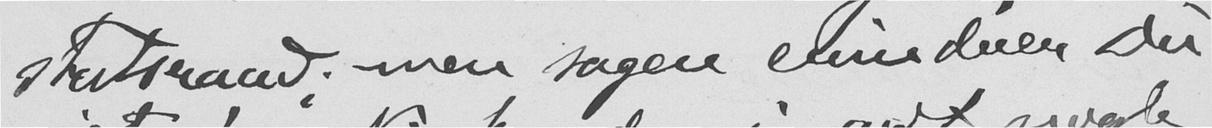statsraad; men sagen erindrer Du
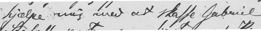hjælpe mig med at skaffe Gabriel
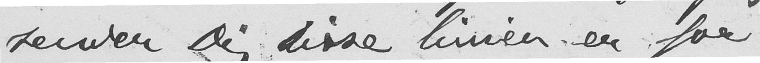sender Dig disse linier er for-
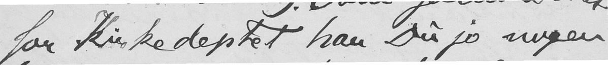for Kirkedeptet har Du jo nogen-
